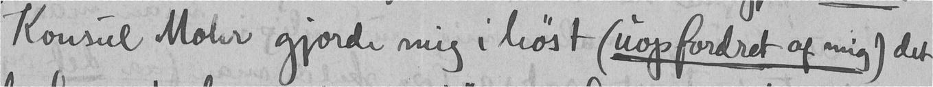Konsul Mohr gjorde mig i høst (uopfordret af mig) det
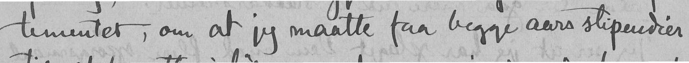tementet, om at jeg maate faa begge aars stipendier
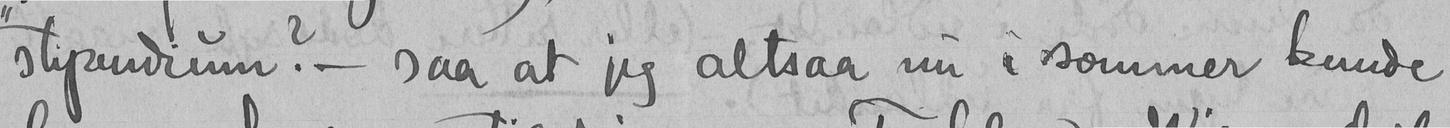stipendium? - saa at jeg altsaa nu i sommer kunde
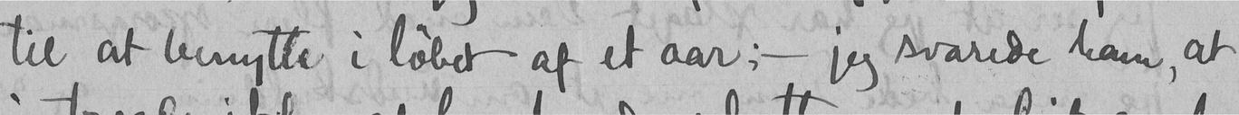til at benytte i løbet af et aar; - jeg svarede ham, at
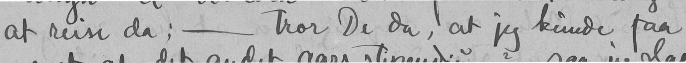at reise da; - tror De da, at jeg kunde faa
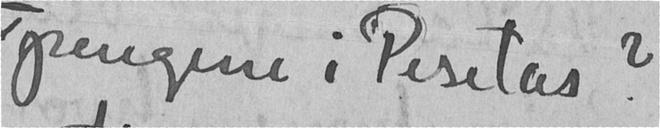pengene i Pesetas?
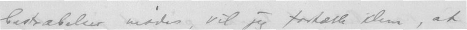bestræbelser mødes, vil jeg fortælle Dem, at
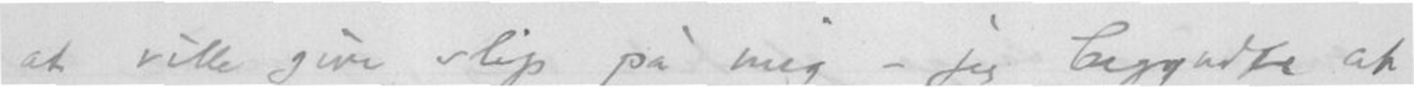at ville give slip på mig - jeg begyndte at
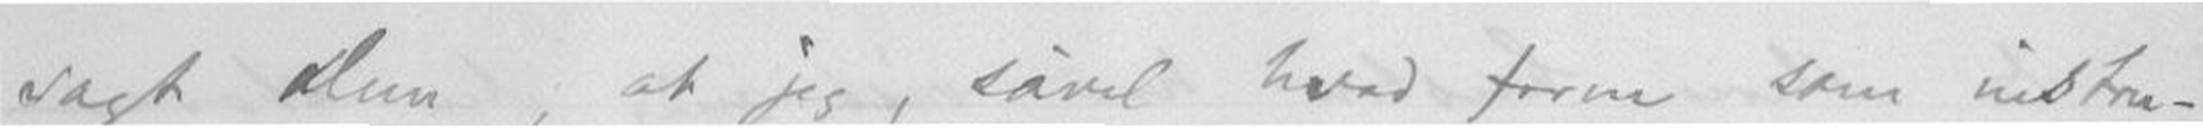sagt Dem; at jeg, såvel hvad form som instru-
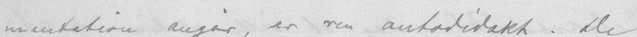mentation angår, er ren autodidakt. De
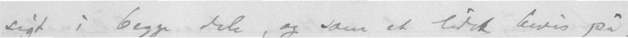sigt i begge dele, og som et lidet bevis på,
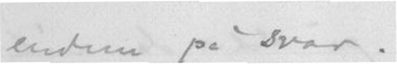endnu på svar.
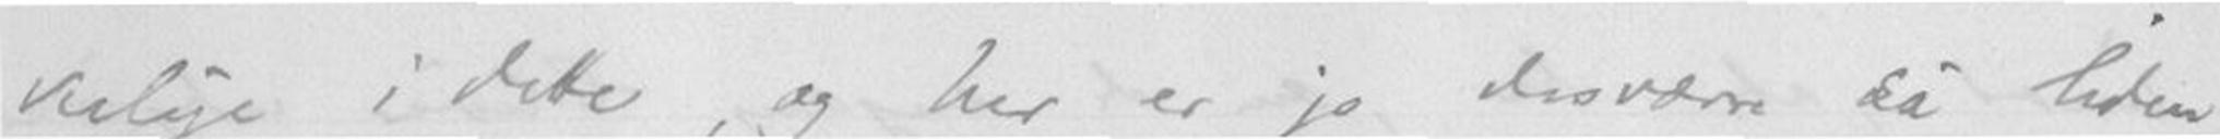kelige i dette, og her er jo desværre så liden
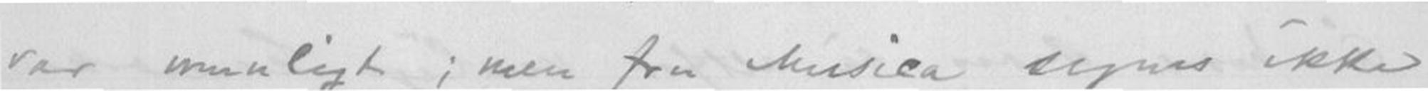var muligt; men fru? Musica synes ikke
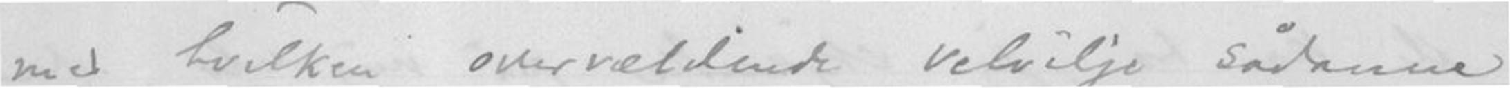med hvilken overvældende velvilje sådanne
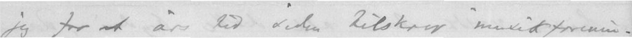jeg for et års siden tilskrev "musikforenin-
